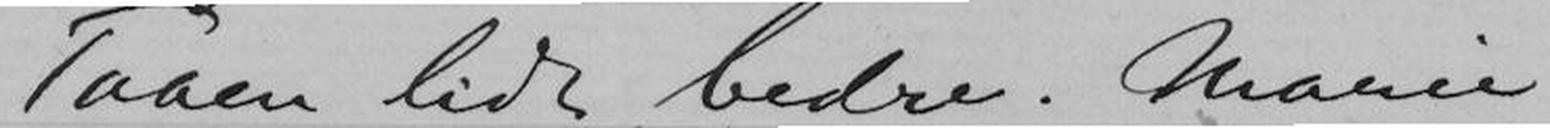Taaen lidt bedre. Marie
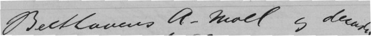Beethovens A-moll og desuden
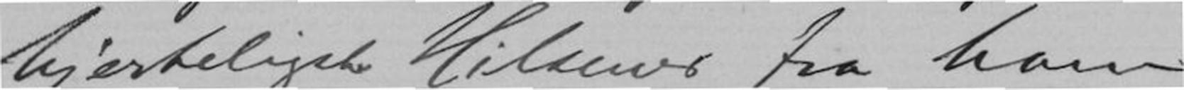hjerteligste Hilsener fra ham
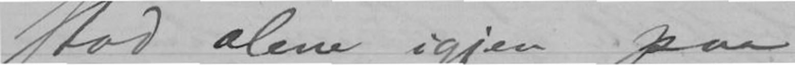stod alene igjen paa
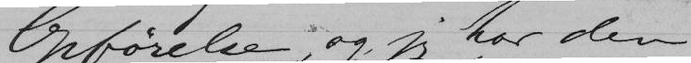Opførelse, og jeg har den
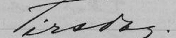Tirsdag.
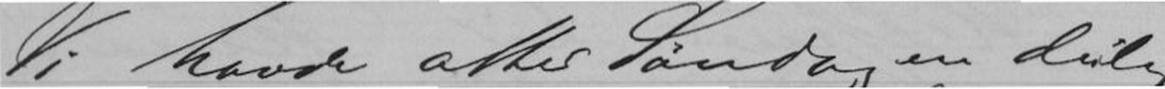Vi havde atter Søndag en deilig
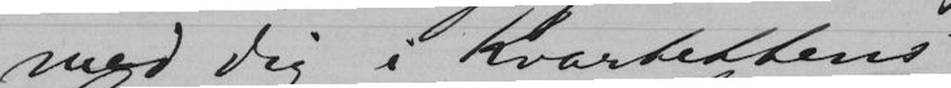med Dig i Kvartettens
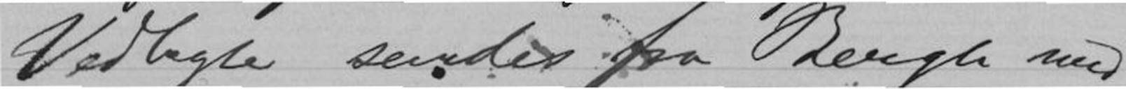Vedlagte sendes fra Bergh med
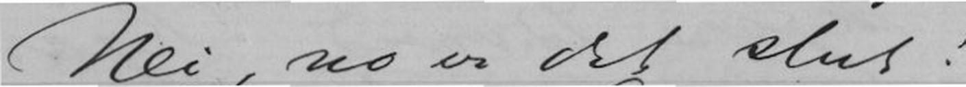Nei, no er det slut!
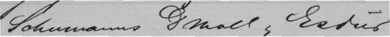- Schumanns D moll, Esdur
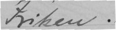Friken. -
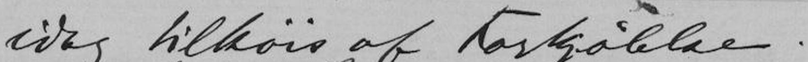idag tilkøis af Forkjølelse.
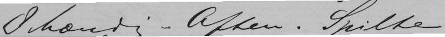8 hændig-Aften. Spilte
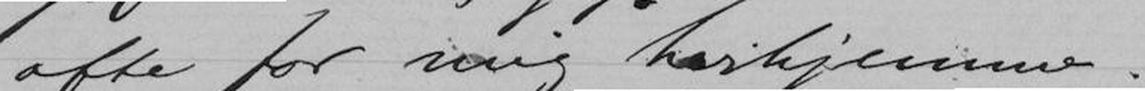ofte for mig herhjemme.
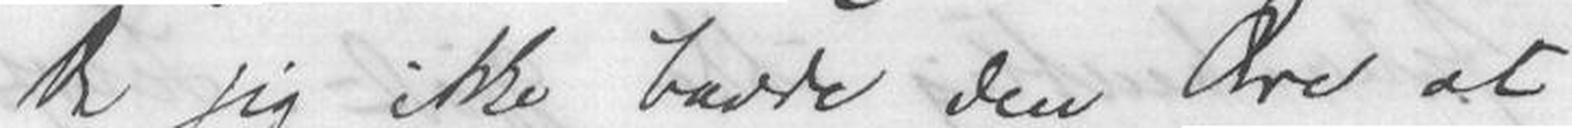Da jeg ikke hadde den Ære at
